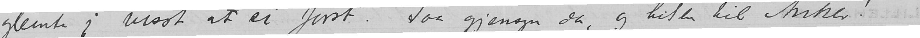glemte jeg visst at si først. Paa gjensyn du, og hilsen til Anker!
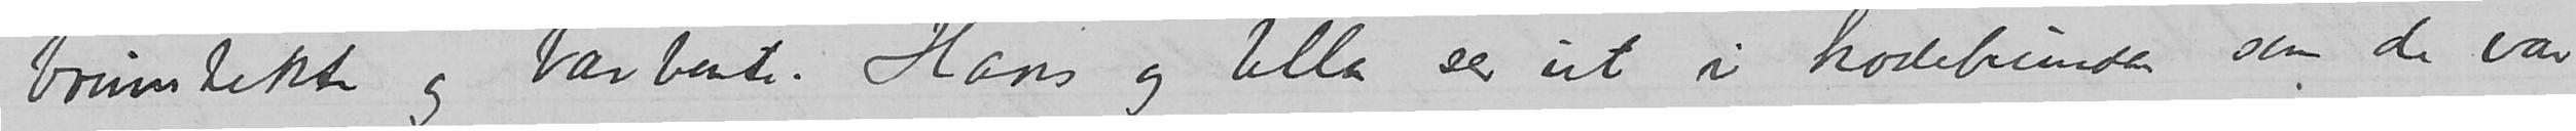brunstekte og barbente. Hans og Ulla ser ut i hodebunden som de var
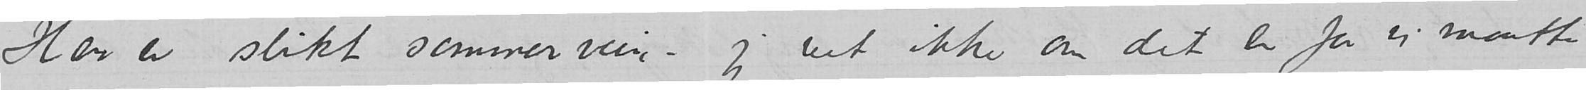Her er slikt sommerveir – jeg vet ikke om det er for vi maatte
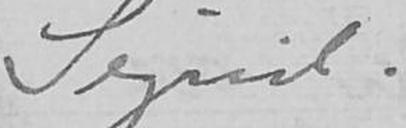Sigrid
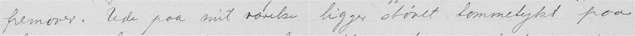fremover. Ude paa mit værelse ligger støvet tommetykt paa
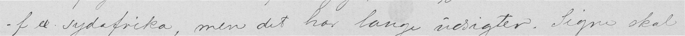- f.ex. sydafrika, men det har lange udsigter. Signe skal
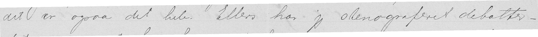det er ogsaa det hele. Ellers har jeg stenograferet debatter -
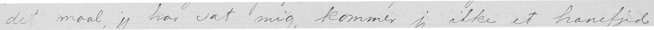det maal, jeg har sat mig, kommer jeg ikke et hanefjed
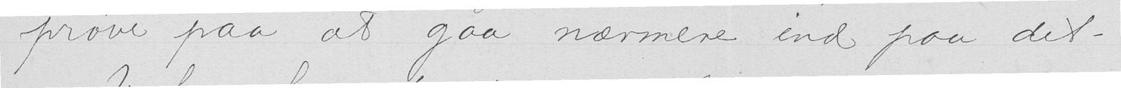prøve paa at gaa nærmere ind paa det -
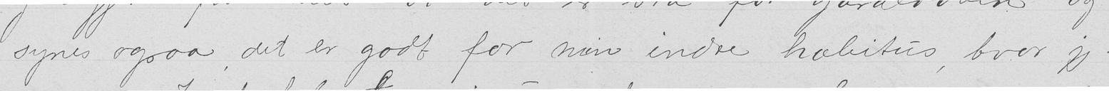synes ogsaa det er godt for min indre habitus, tror jeg.
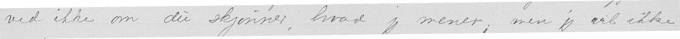ved ikke om du skjønner, hvad jeg mener; men jeg vil ikke
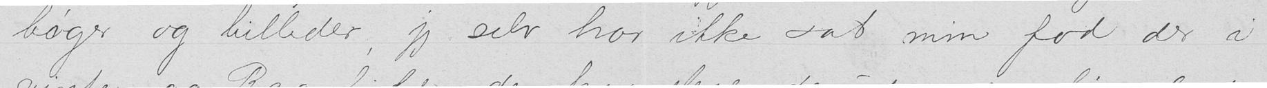bøger og billeder, jeg selv har ikke sat min fod der i
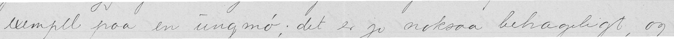exempel paa en ungmø; det er jo noksaa behageligt, og
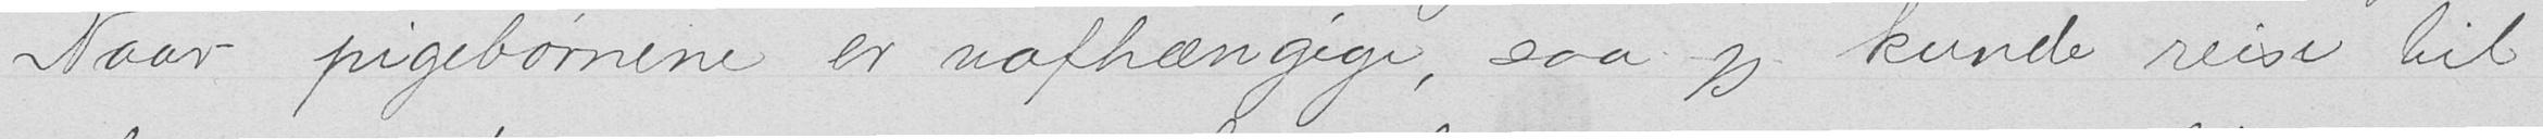Naar pigebørnene er uafhængige, saa jeg kunde reise til
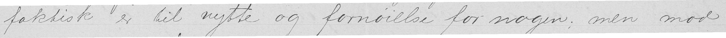faktisk er til nytte og fornøielse for nogen; men mod
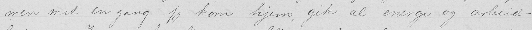men med en gang jeg kom hjem, gik al energi og arbeids-
